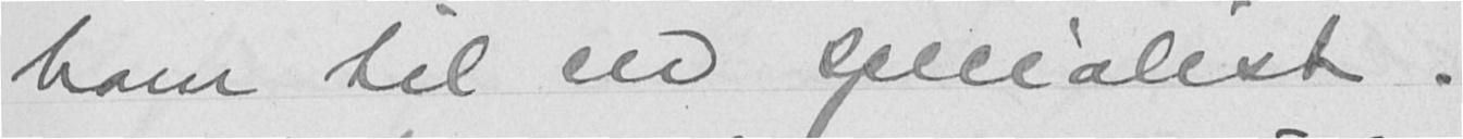ham til en specialist.
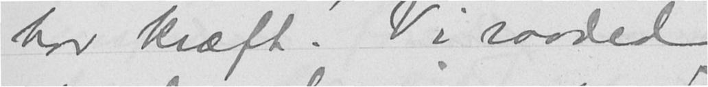har kræft. Vi raaded
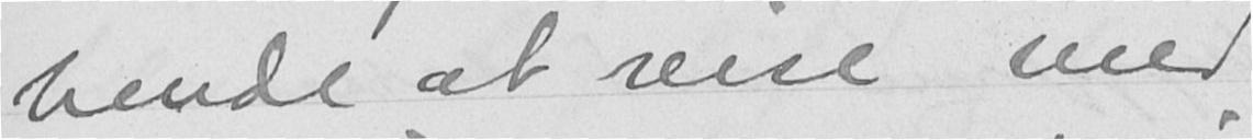hende at reise med
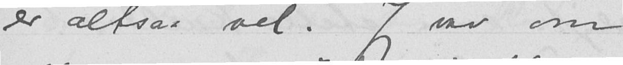er altsaa vel. Jeg var om
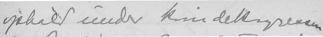ophold under kvindekongressen
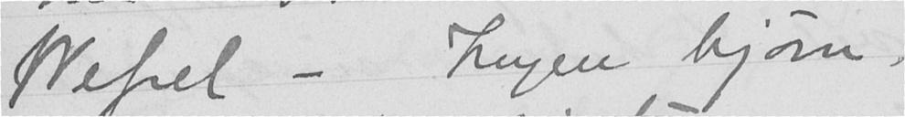Wessel - Ingen bjørn,
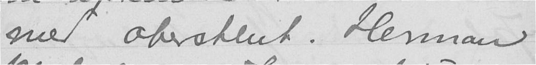med oberstlnt. Herman
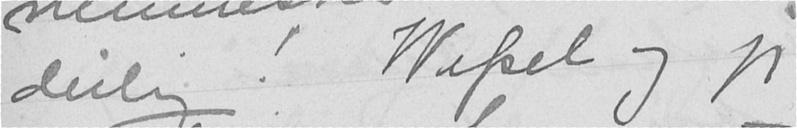deilig! Wessel og jeg
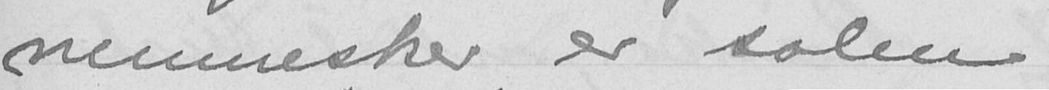mennesker er solen
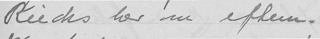Riecks her om efterm.
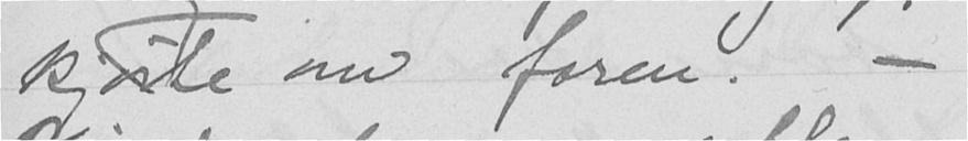kjørte om form. -
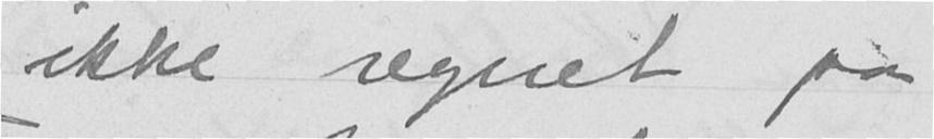ikke regnet paa
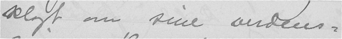klogt om sine verdens-
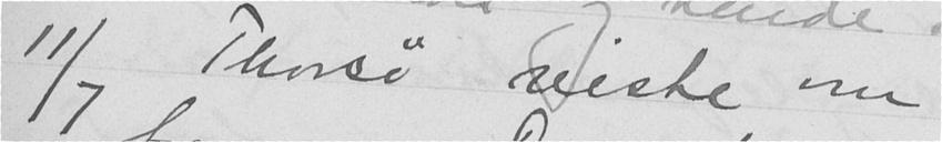11/7 Thorsø reiste om
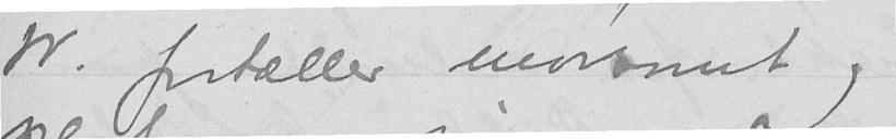W. fortæller morsomt og
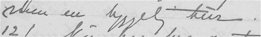men en hyggelig tur.
